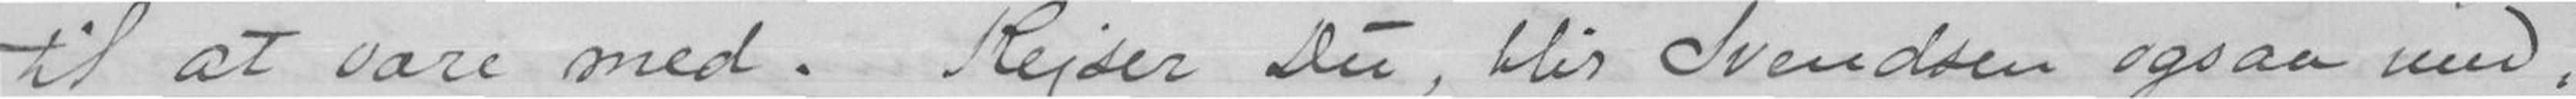til at være med. Rejser Du, blir Svendsen ogsaa med.
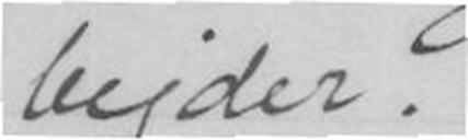bejder.
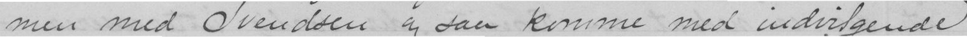men med Svendsen og saa komme med indvilgende
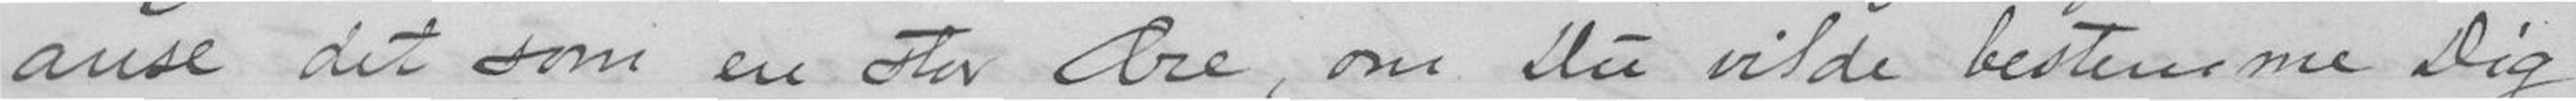anse det som en stor Ære, om Du vilde bestemme Dig
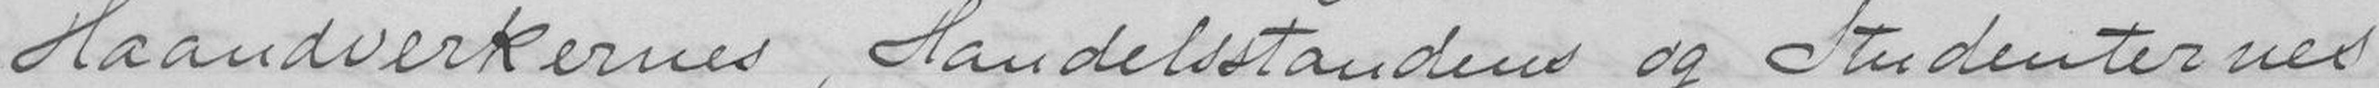Haandverkernes, Handelsstandens og Studenternes
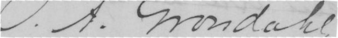O.A.Grøndahl
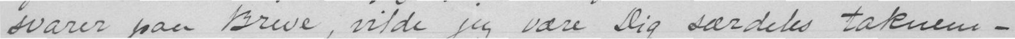Svarer paa Breve, vilde jeg være Dig særdeles taknem-
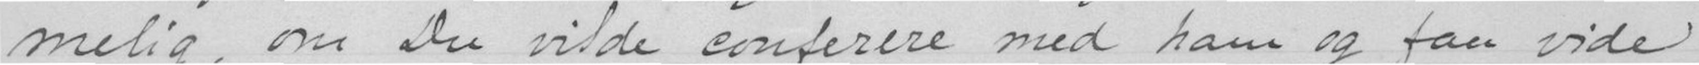melig, om Du vilde conferere med ham og for vide
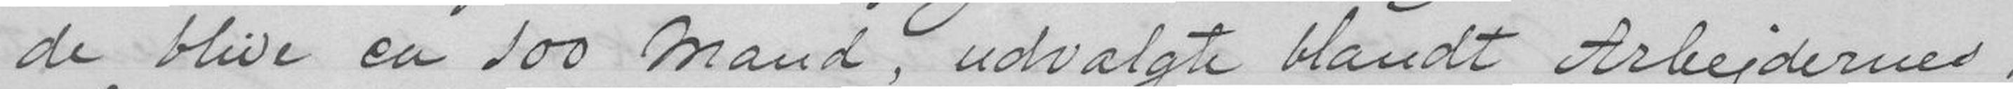de blive ca 100 mand, udvalgte blandt Arbejdernes,
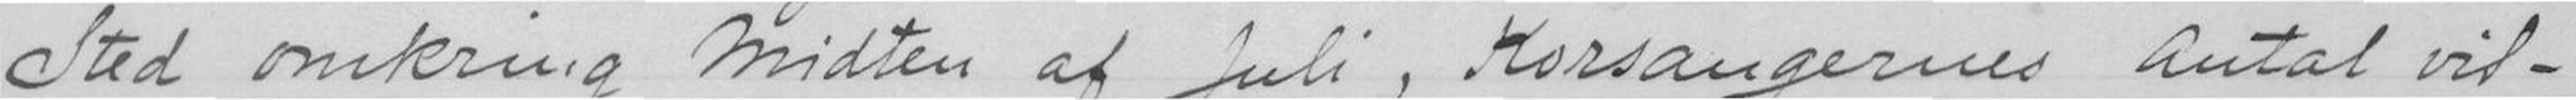Sted omkring Midten af Juli, Korsangernes antal vil-
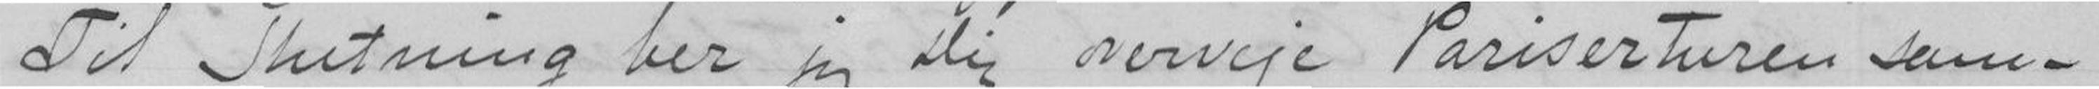Til Slutning ber jeg Dig overveje Pariserturen sam-
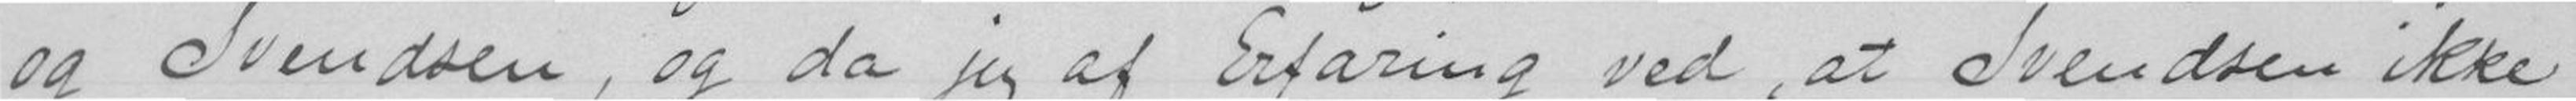og Svendsen, og da jeg af Erfaring ved , at Svendsen ikke
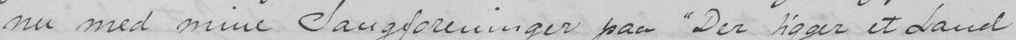nu med mine Sangforeninger paa "Der ligger et land
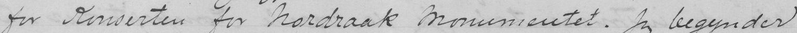for Konserten for nordraak monumentet. Jeg begynder
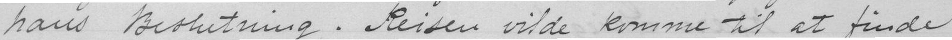hans Beslutning. Reisen vilde komme til at finde
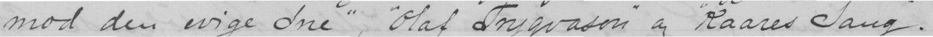mod den evige Sne", "Olaf Trygvarson" og "Kaares Sang"
